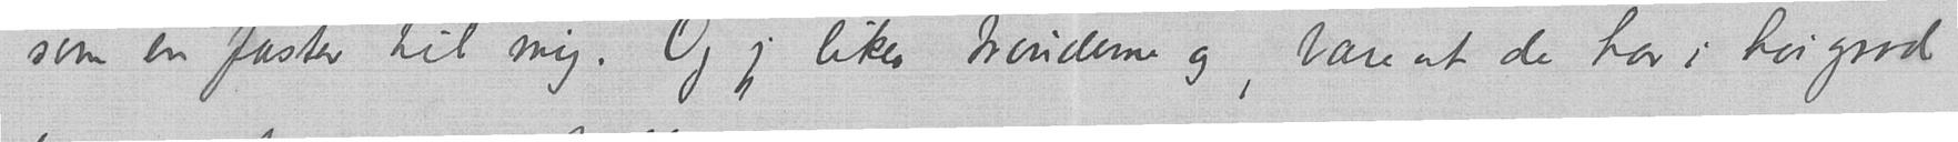som en faster til mig. Og jeg likes trønderne og, bare at de har i høi grad
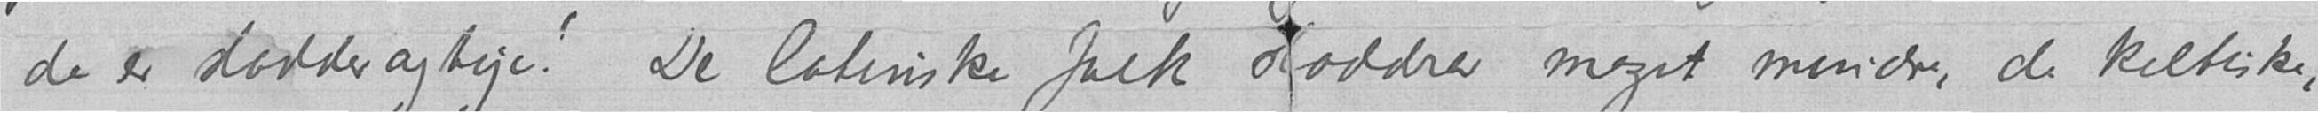de er sladderagtige! De latinske folk sladdrer meget mindre, de keltiske,
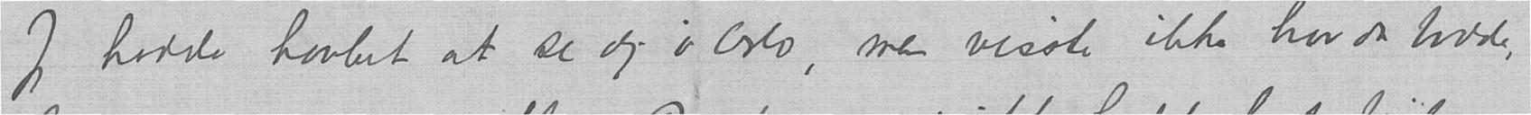Jeg hadde haabet at se dig i Oslo, men visste ikke hvor du bodde,
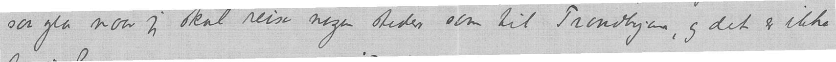saa gla naar jeg skal reise nogen steder som til Trondhjem, og det er ikke
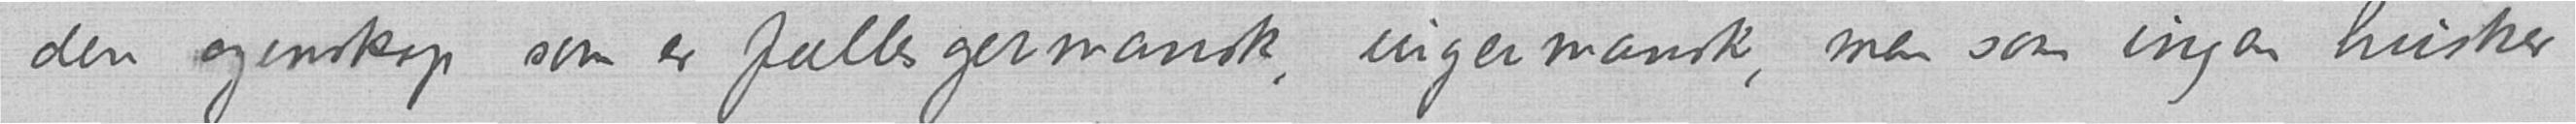den egenskap som er fællesgermansk, urgermansk, men som ingen husker
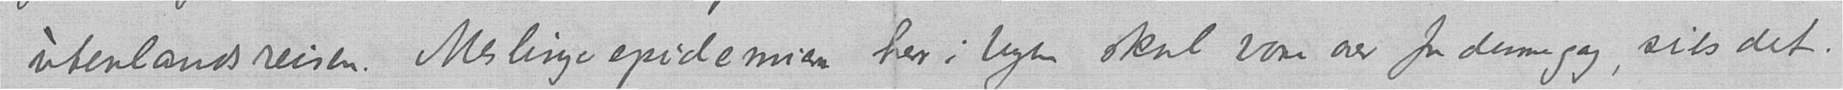utenlandsreisen. Meslingeepidemien her i byen skal være over for denne gang, sies det.
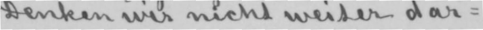Denken wir nicht weiter dar-
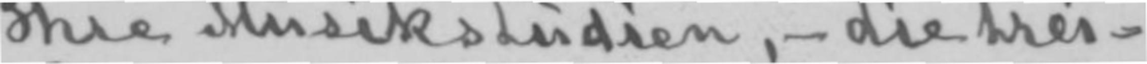Ihre Musikstudien,- die trei-
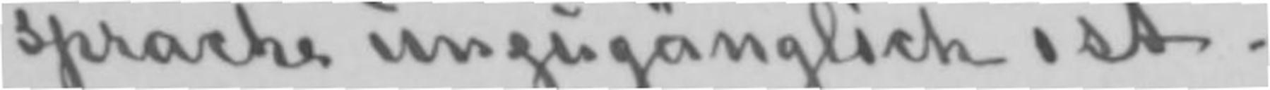sprache unzugänglich ist.
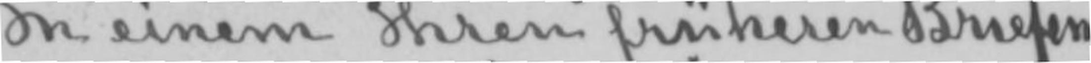In einem Ihren früheren Briefen
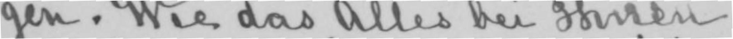gen. Wie das Alles bei Ihnen
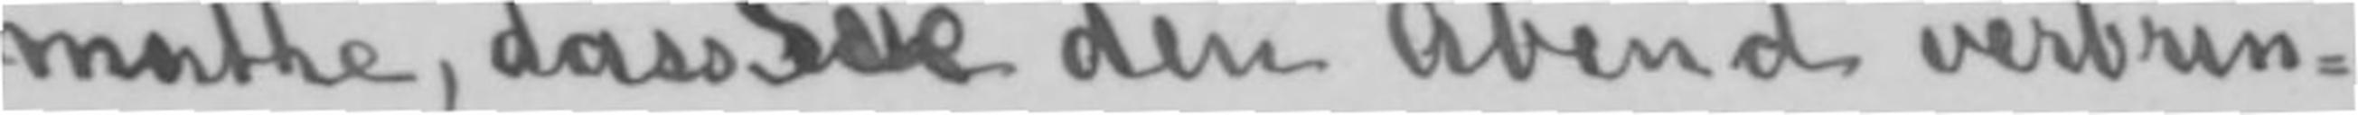muthe, dass ichSie den Abend verbrin-
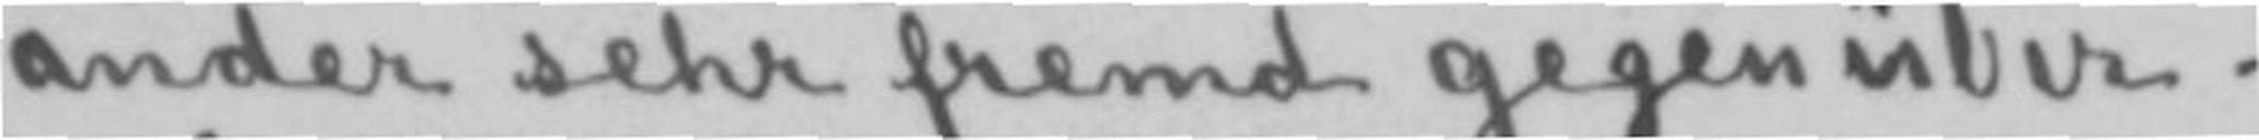ander sehr fremd gegenüber.
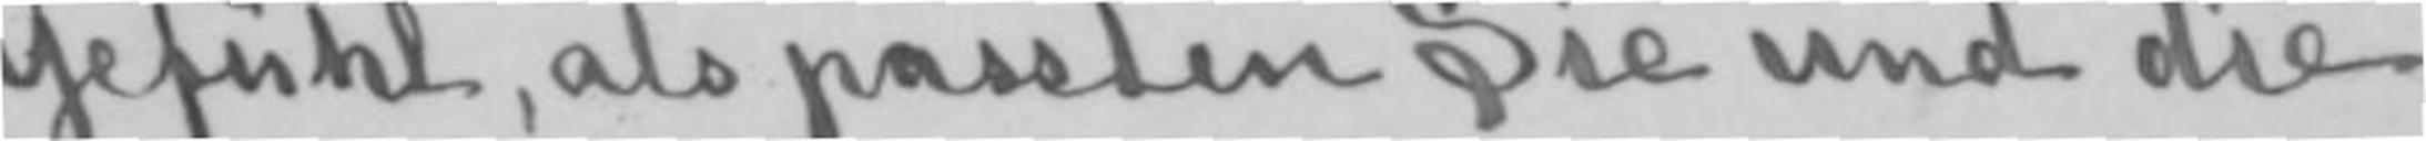Gefühl, als passten Sie und die
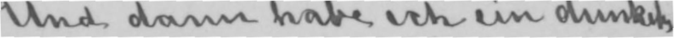Und dann habe ich ein dunkeles
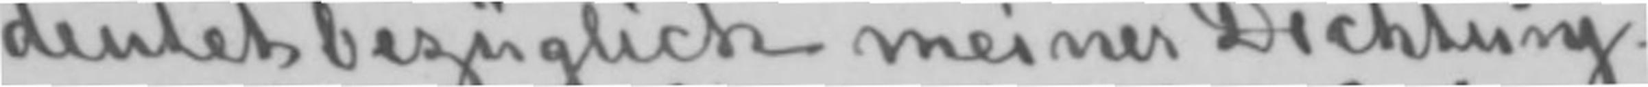deutet bezüglich meiner Dichtung
